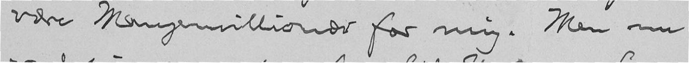være Mangemillionær for mig. Men nu
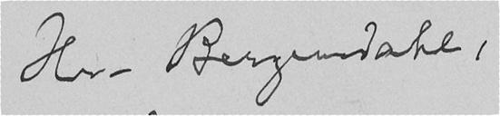Hr. Bergendahl,
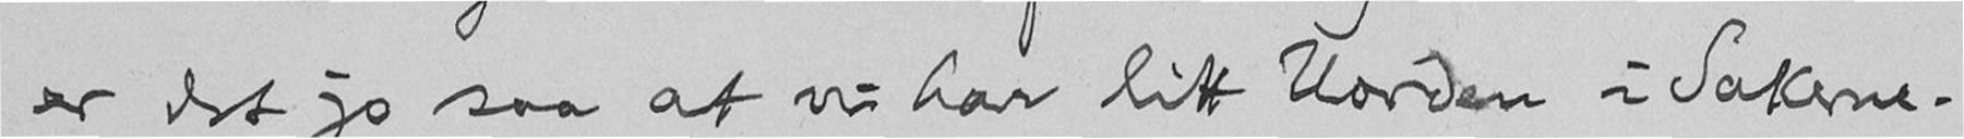er det jo saa at vi har litt Uorden i Sakerne.
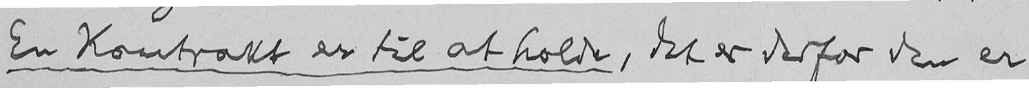En Kontrakt er til at holde, det er derfor den er
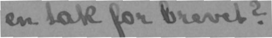en tak for brevet?
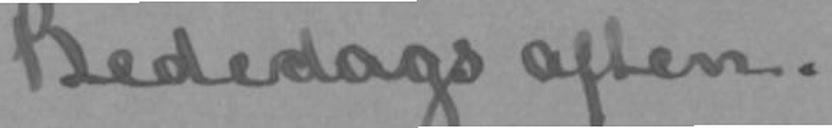Bededags aften.
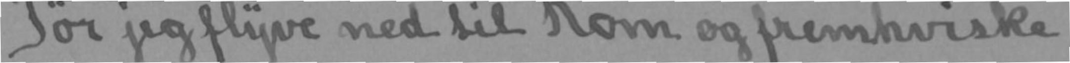Tør jeg flyve ned til Rom og fremhviske
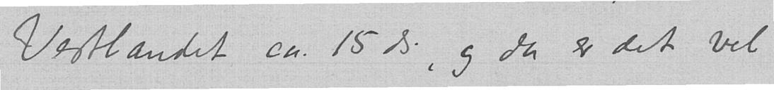Vestlandet ca. 15 ds., og da er det vel
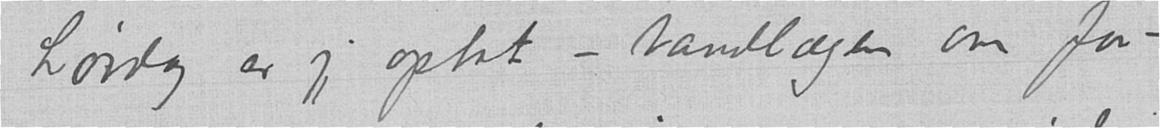Lørdag er jeg optat – tandlægen om for-
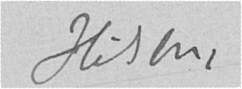Hilsen,
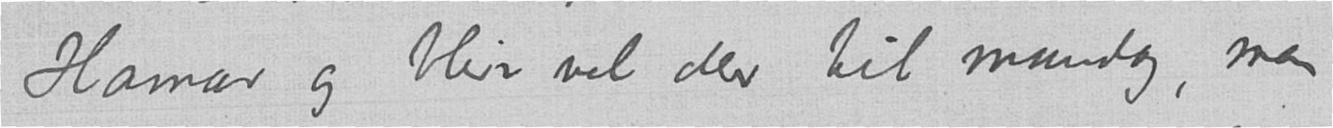Hamar og blir vel der til mandag, men
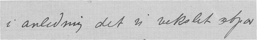i anledning det vi vekslet etpar
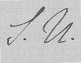S.U.
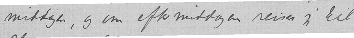middagen, og om eftermiddagen reiser jeg til
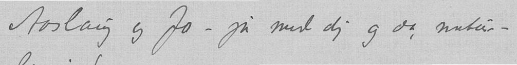Aaslaug og Jo – ja med dig og da natur-
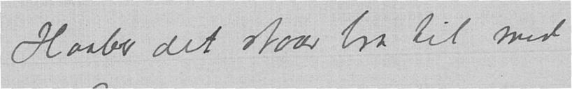Haaber det staar bra til med
